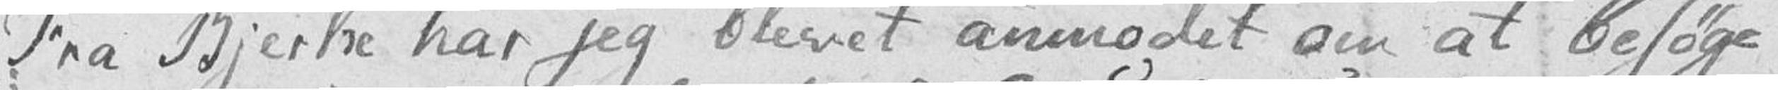Fra Bjerke har jeg blevet anmodet om at besøge
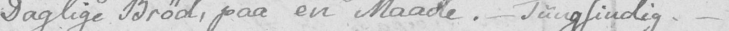Daglige Brød, paa en Maade. – Tungsindig. –
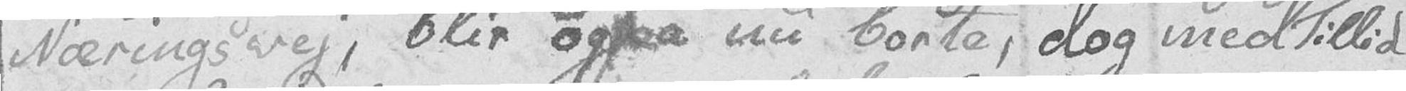Næringsvej, blir ogsaa nu borte, dog med Tillid
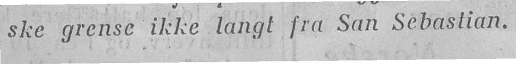ske grense ikke langt fra San Sebastian
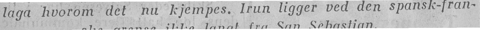laga hvorom det nu kjempes. Irun ligger ved den spansk-fran-
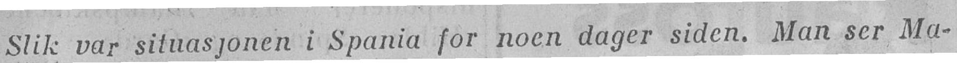Slik var situasjonen i Spania for noen dager siden. Man ser Ma-
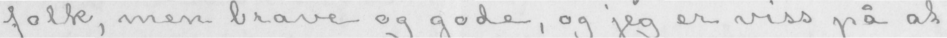folk, men brave og gode, og jeg er viss på at
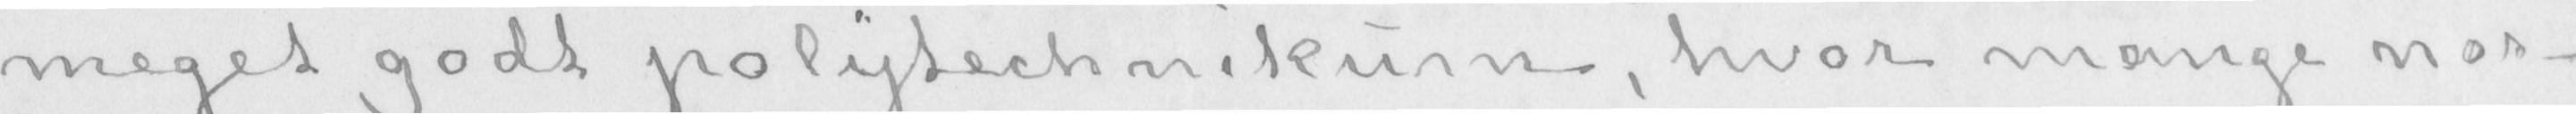meget godt polytechnikum, hvor mange nor-
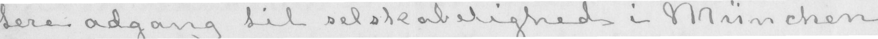tere adgang til selskabelighed i München
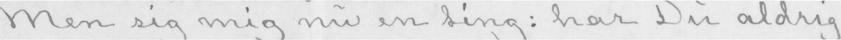Men sig mig nu en ting: har Du aldrig
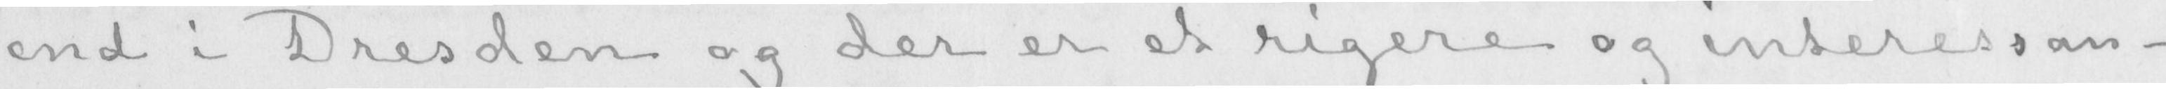end i Dresden og der er et rigere og interessan-
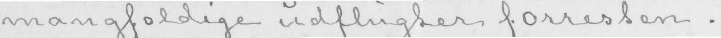mangfoldige udflugter forresten.-
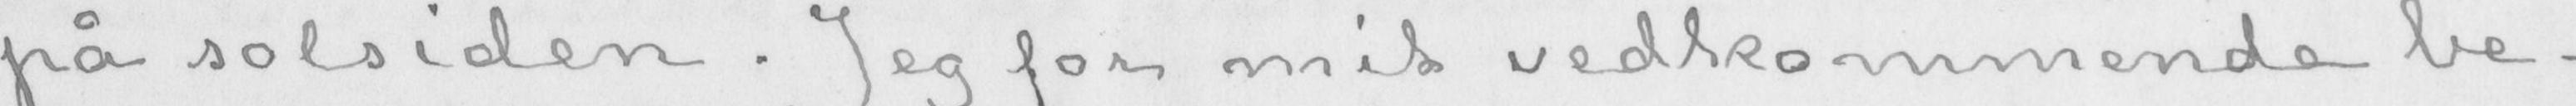på solsiden. Jeg for mit vedkommende be-
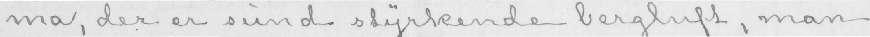ma, der er sund styrkende bergluft, man
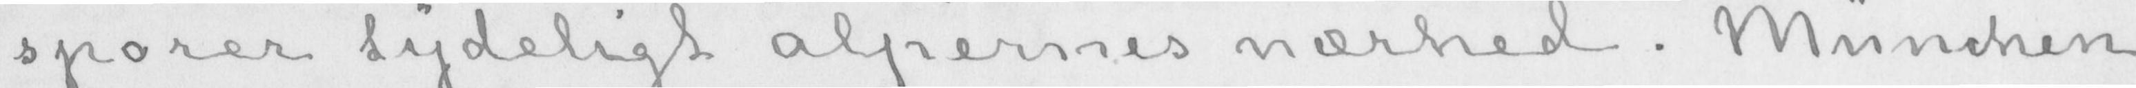sporer tydeligt alpernes nærhed. München
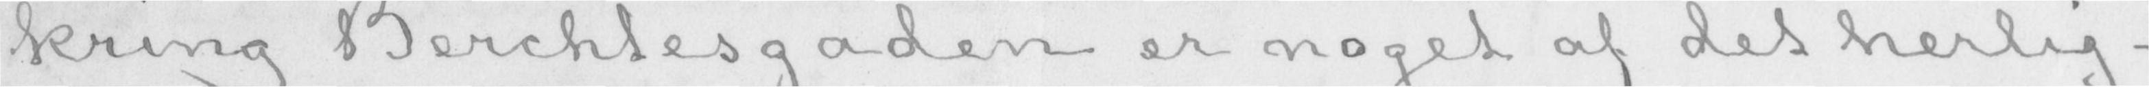kring Berchtesgaden er noget af det herlig-
